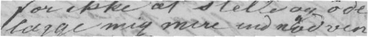lægge mig mere end nødven
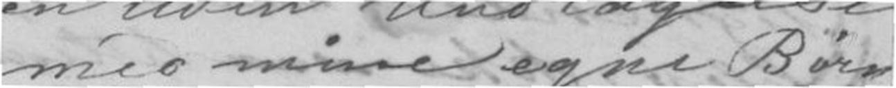med mine egne Børn,
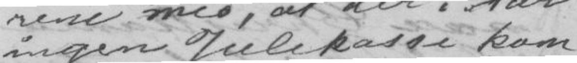ingen Julekasse kom
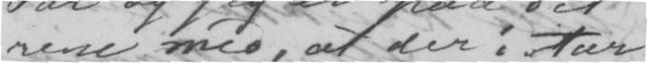rene med, at der i Aar
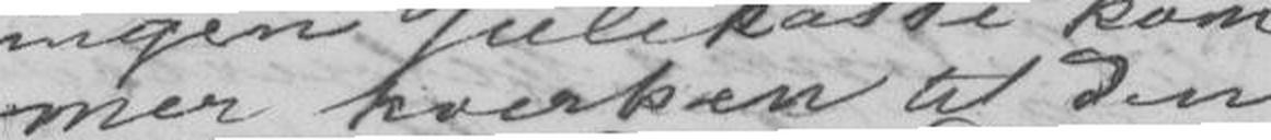mer hverken til Den
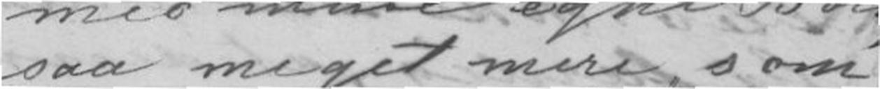saa meget mere, som
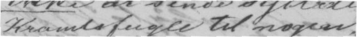Kramlefugle til nogen
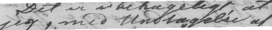jeg, med Undtagelse af
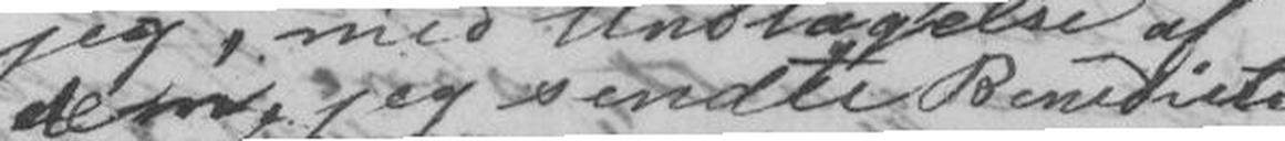dem, jeg sendte Benedicte
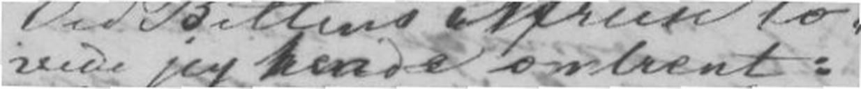vede jeg hende omtrent:
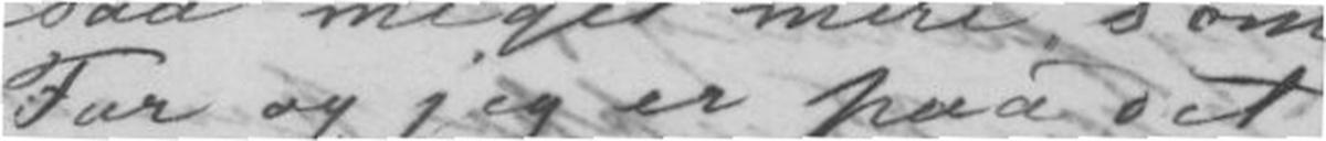Far og jeg er paa det
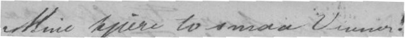Mine kjære to smaa Venner!
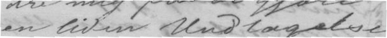en liden Undtagelse
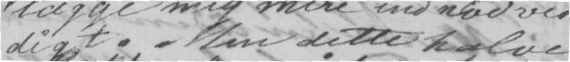digt. Men dette halve
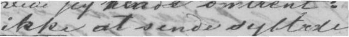ikke at sende syltæde
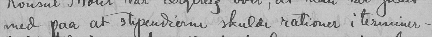med paa at stipendierne skulde rationer i terminer -
